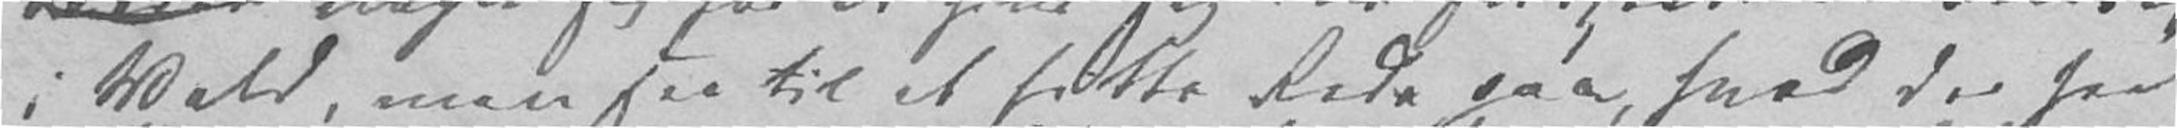i Vold, men see til at fatte Rede paa, hvad der fra
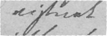vistnok
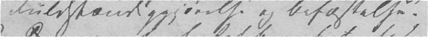Fuldstændiggjørelse og Befæstelse.
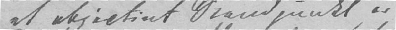et objectivt Standpunkt er
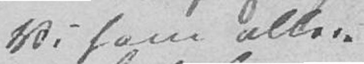Vi have allere-
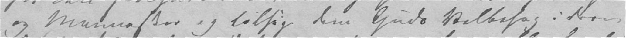og Mennesker og tilsige dem Guds Velbehag i deres
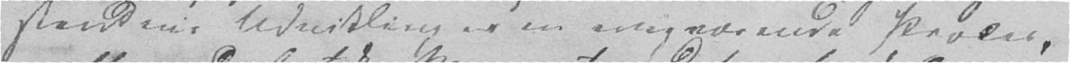standens Udvikling er en evigvarende Proces.
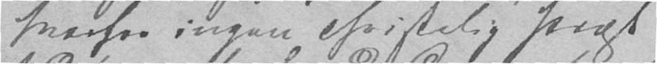hvorfor ingen christelig Præst
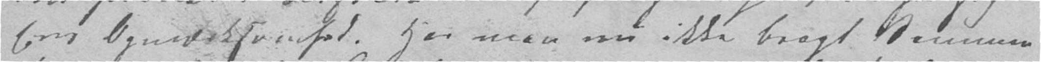Ens Opmærsomhed. Har man nu ikke bragt Sammen-
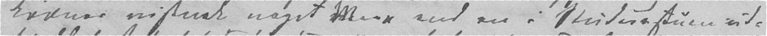kræves vistnok noget Mere end en i Studiestuen ud-
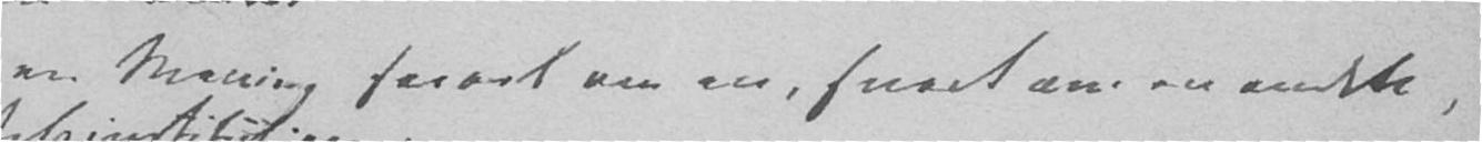en Mening snart om en, snart om en anden,
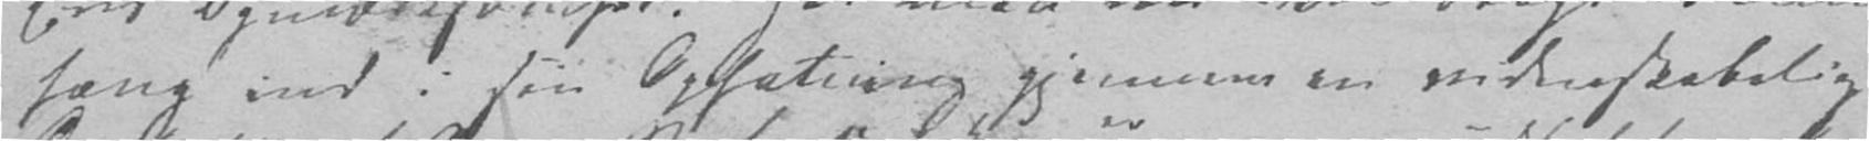hæng ind i sin Opfatning gjennem en videnskabelig
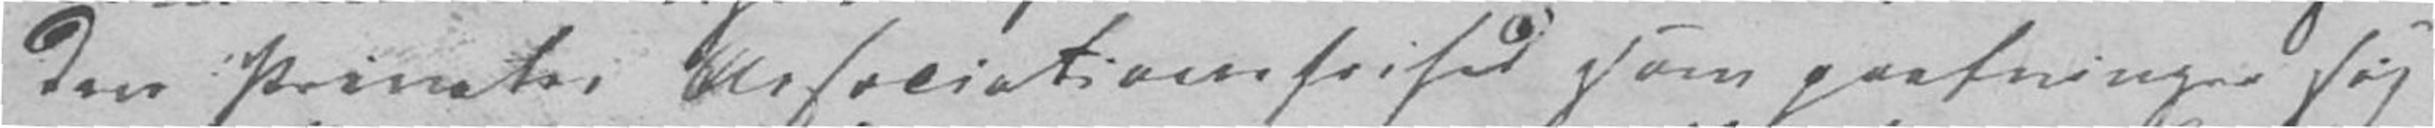den Privates Associationsfrihed som paatvinger sig
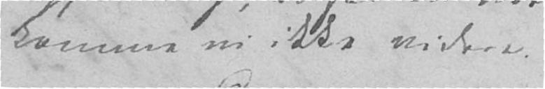komme vi ikke videre.
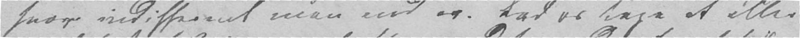hvor indifferent man end er. Lad os tage et eller
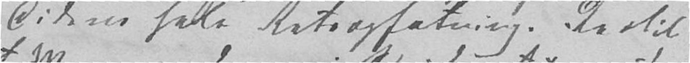Tidens hele Retsopfatning. Dertil
